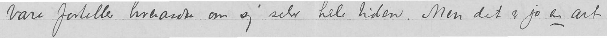bare forteller hverandte om sig selv hele tiden. Men det er jo en art
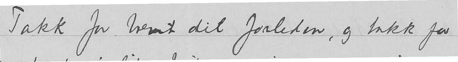Takk for brevet dit forleden, og takk for
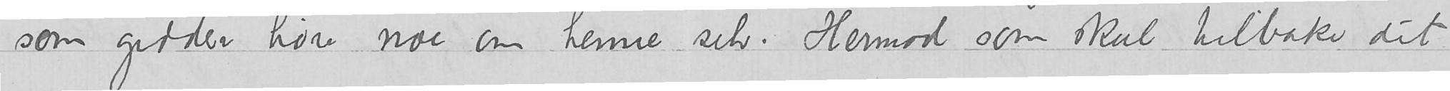som gidder høre noe om henne selv. Hermod som skal tilbake dit
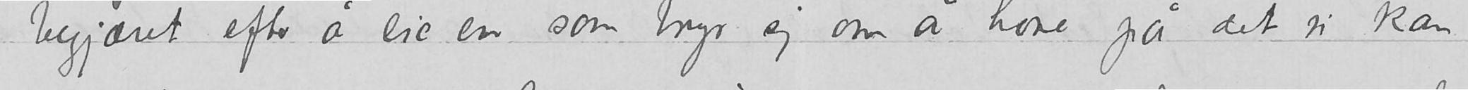begjæret efter å eie en som bryr sig om å høre på det vi kan
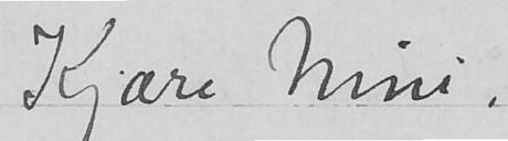Kjære Nini,
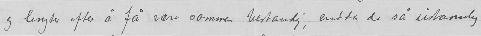og lengter efter å få være sammen bestandig, endda de så ustanselig
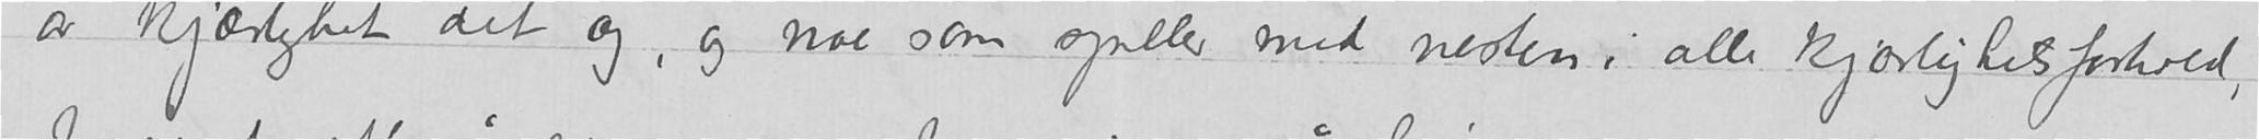av kjærlighet det og, og noe som spiller med nesten i alle kjærlighetsforhold,
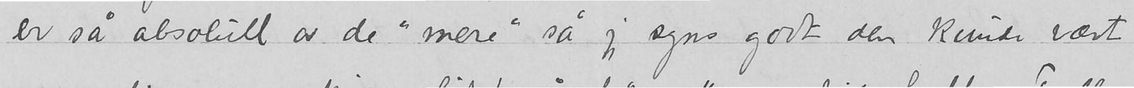er sa absolutt av de «mere» så jeg syns godt dem kunde vært
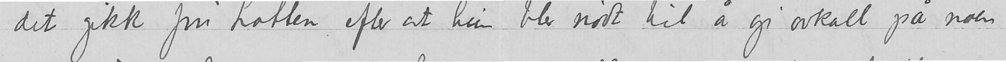det gikk fru Lotten, efter at hun blev nødt til å gi avkall på noen
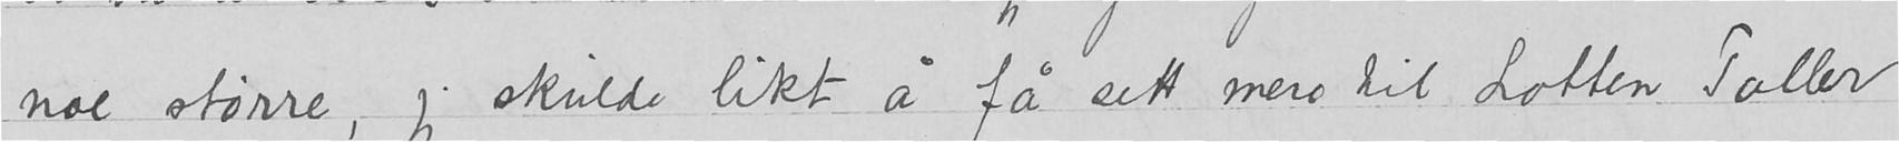noe større, jeg skulde likt å få sett mere til Lotten Toller
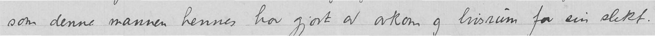som denne mannen hennes har gjort av avkom og livsrum for sin slekt.
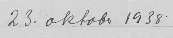23. oktober 1938.
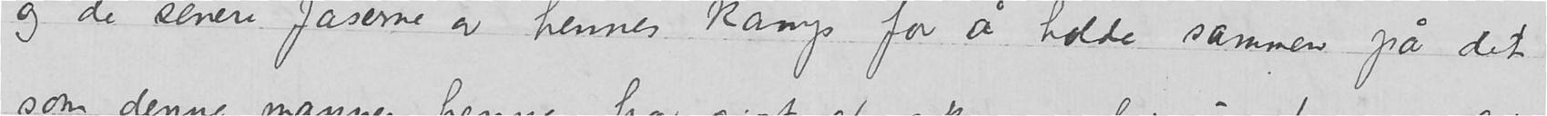og de senere faserne av hennes kamp for å holde sammen på det
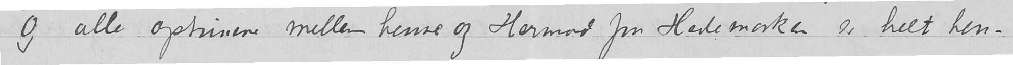Og alle optrinene mellem henne og Hermod fra Hædemarken er helt hen-
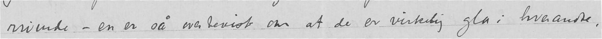rivende – en er så overbevist om at de er virkelig gla i hverandre,
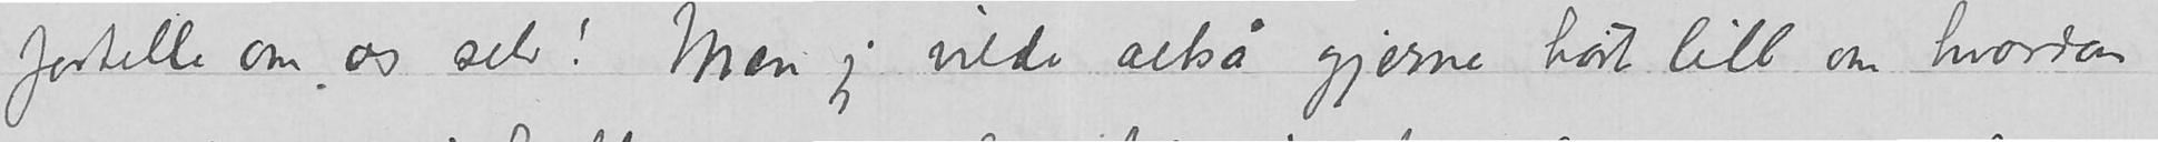fortelle om os selv! Men jeg vilde altså gjerne hørt litt om hvordan
